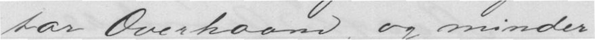tar Overhaand, og minder
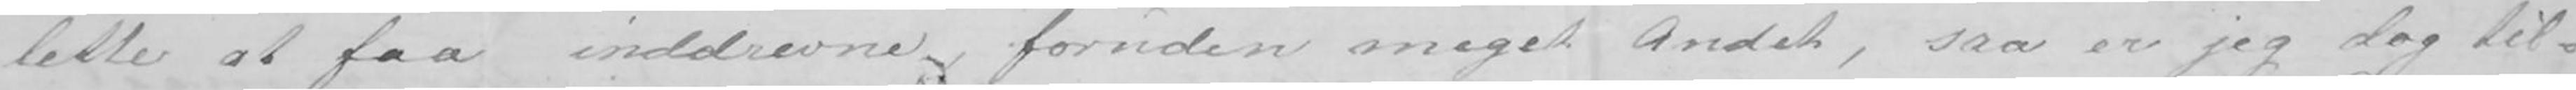lette at faa inddrevne, foruden meget Andet, saa er jeg dog til-
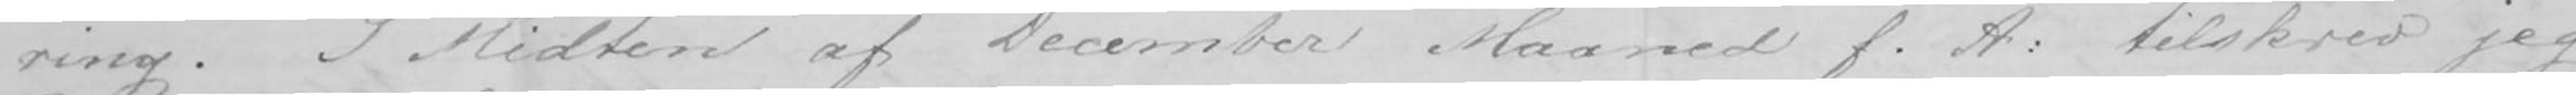ring. I Midten af December Maaned f. A: tilskrev jeg
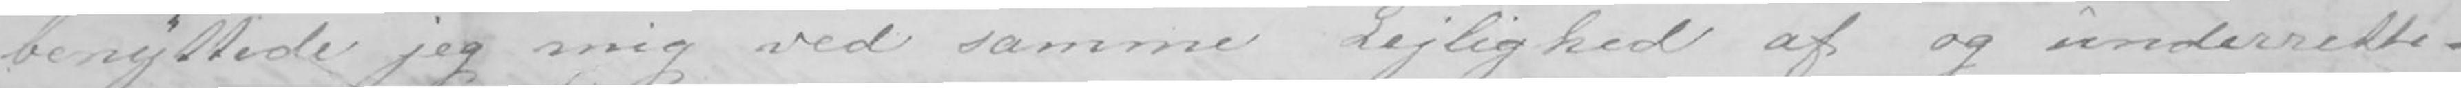benyttede jeg mig ved samme Lejlighed af og underrette-
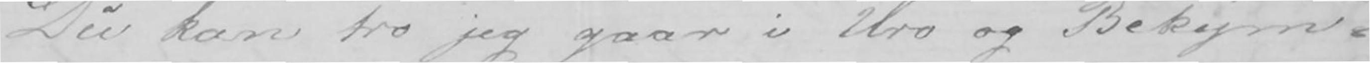Du kan tro jeg gaar i Uro og Bekym-
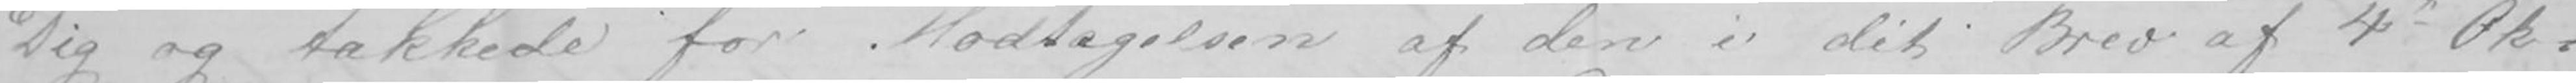Dig og takkede for Modtagelsen af den i dit Brev af 4de Ok-
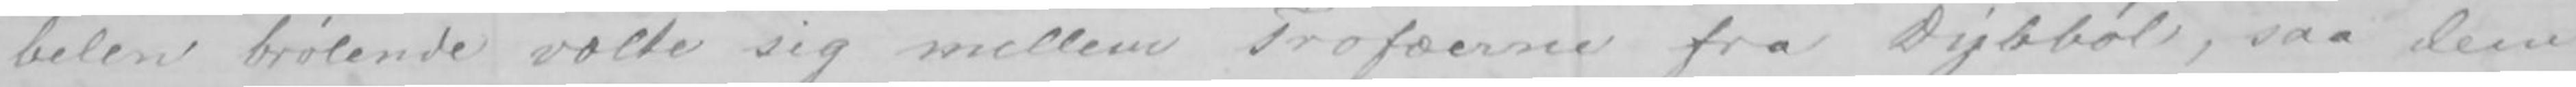belen brølende vælte sig mellem Trofæerne fra Dybbøl, saa dem
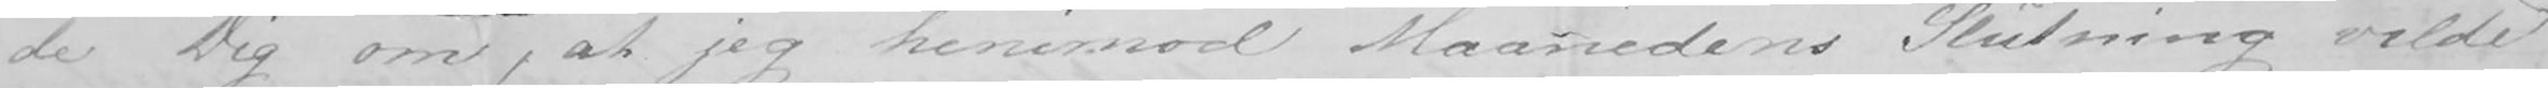de Dig om, at jeg henimod Maanedens Slutning vilde
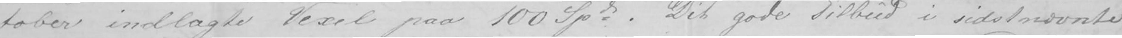tober indlagte Vexel paa 100 Spd. Dit gode Tilbud i sidstnævnte
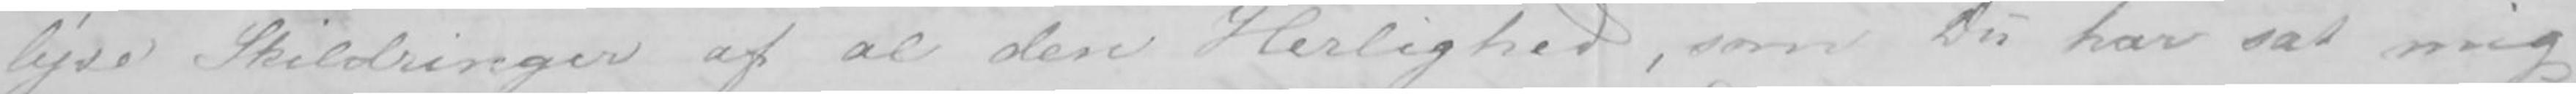lyse Skildringer af al den Herlighed, som Du har sat mig
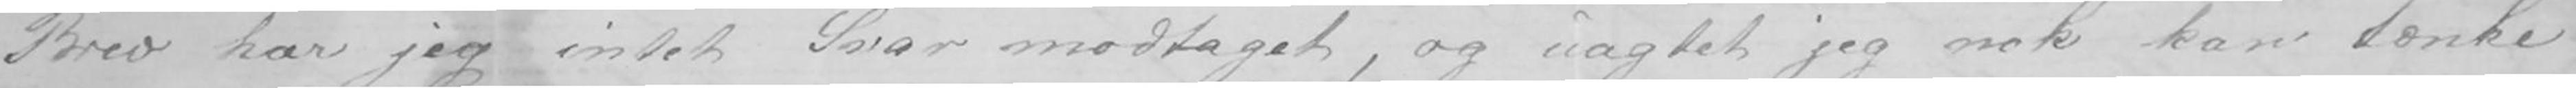Brev har jeg intet Svar modtaget, og uagtet jeg nok kan tænke
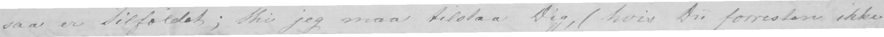saa er Tilfældet; thi jeg maa tilstaa Dig, (hvis Du forresten ikke
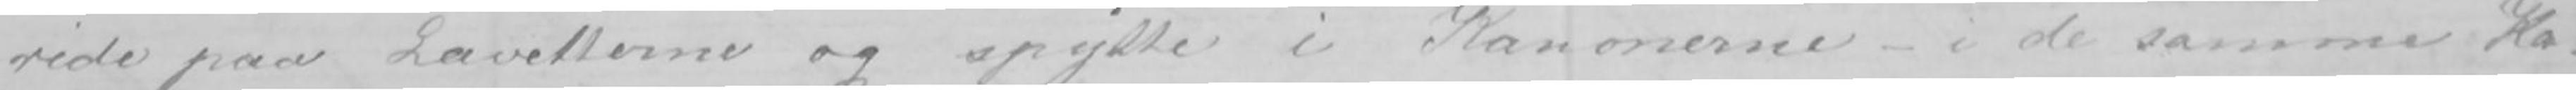ride paa Lavetterne og spytte i Kanonerne- i de samme Ka-
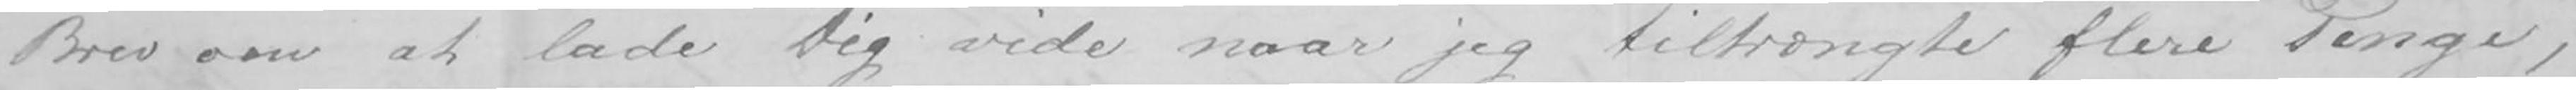Brev om at lade Dig vide naar jeg tiltrængte flere Penge,
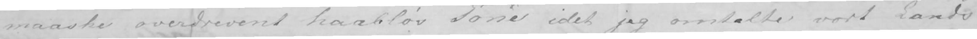maaske overdrevent haabløs Tone idet jeg omtalte vort Lands
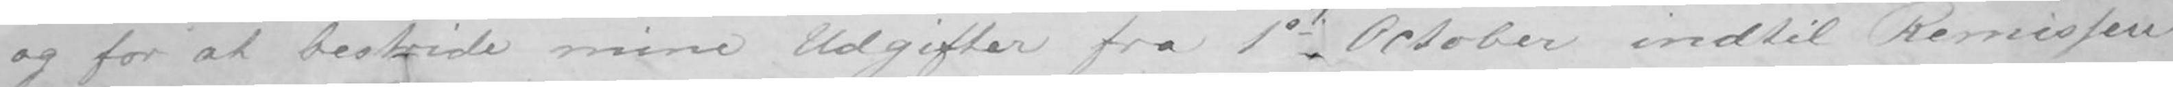og for at bestride mine Udgifter fra 1ste October indtil Remissen
